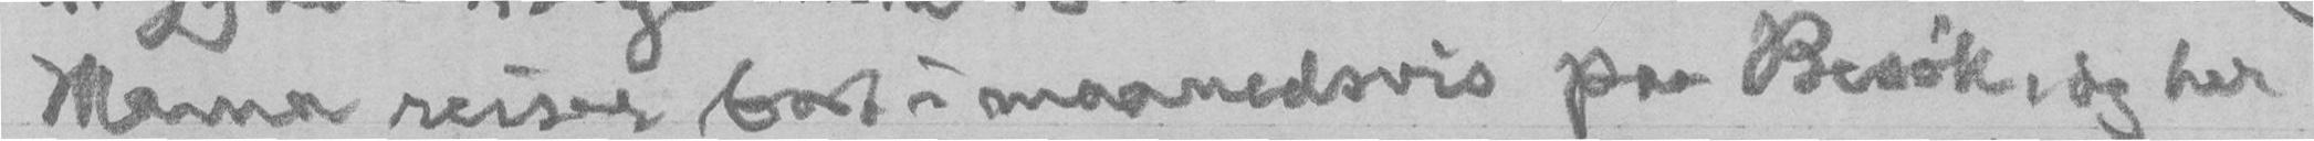Mama reiser bort i maanedsvis paa Besøk, og her
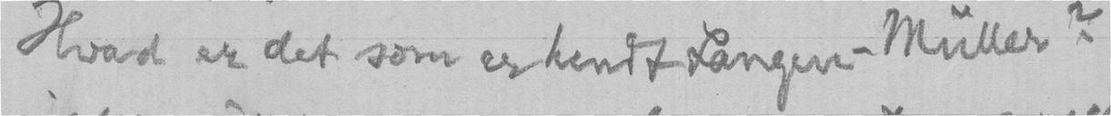Hvad er det som er hendt Langen-Müller?
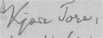Kjære Tore,
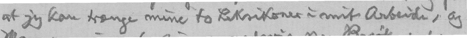at jeg kan trænge mine to Leksikoner i mit Arbeide, og
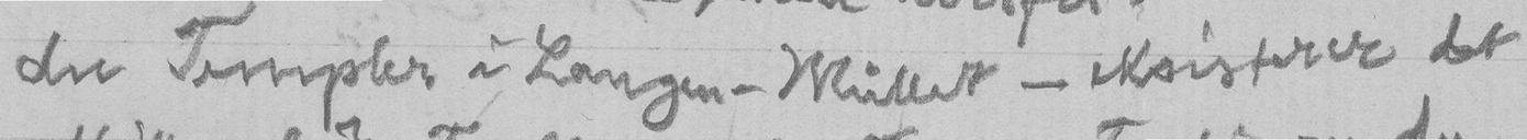du Terrepler i Langen-Müller - eksisterer det
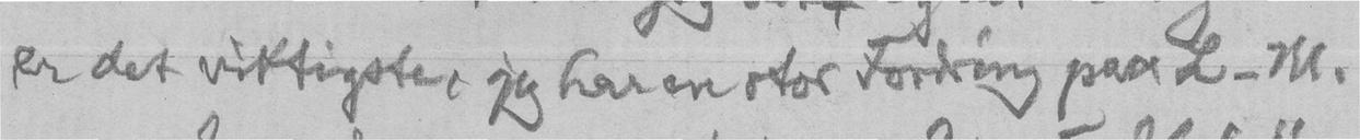er det viktigste, jeg har en stor Fordring paa L-M.
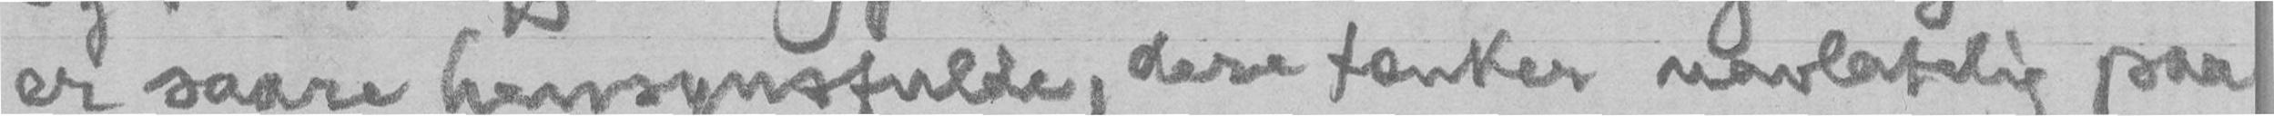er saare hensynsfulde, dere tænker uavlatelig paa
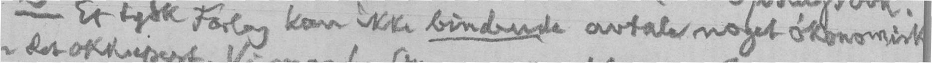- Et tysk Forlag kan ikke bindende avtale noget økonomisk
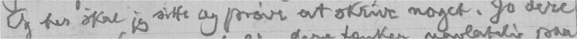Og her skal jeg sitte og prøve at skrive noget. Jo dere
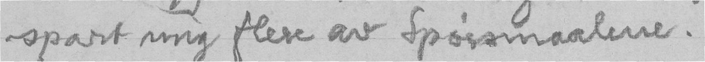spart mig flere av Spørsmaalene.
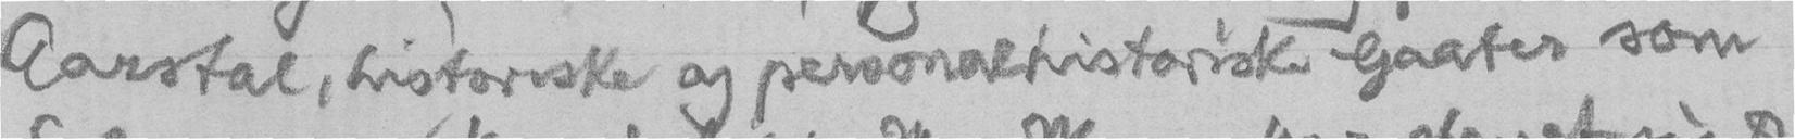Aarstal, historiske og personalhistoriske Gaater som
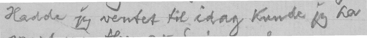Hadde jeg ventet til idag kunde jeg ha
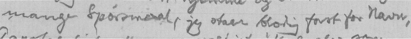mange Spørsmaal, jeg staar blodig fast for Navn,
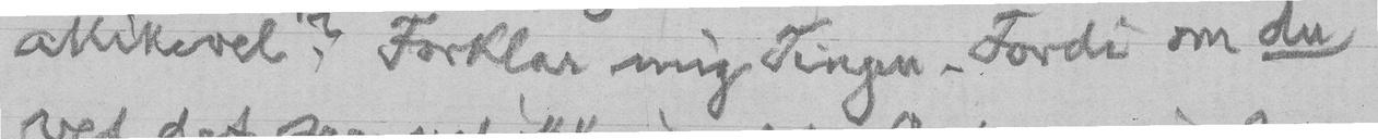allikevel? Forklar mig Tingen. Fordi om du
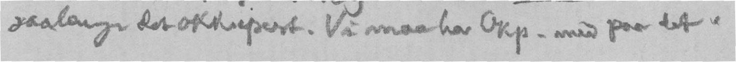saalænge det okkupert. Vi maa ha Okp. med paa det.
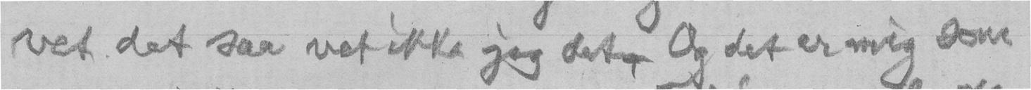vet det saa vet ikke jeg det. Og det er mig som
